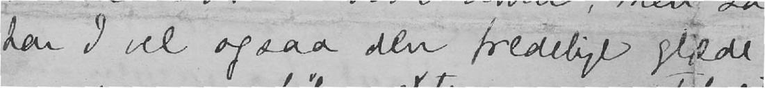har I vel ogsaa den fredelige glæde
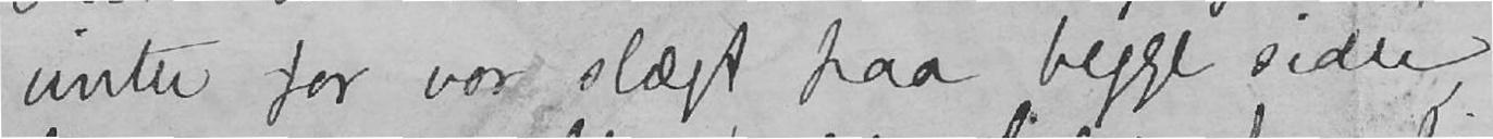vinter for vor slægt paa begge sider,
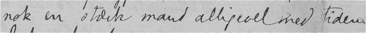nok en stærk mand alligevel med tiden.
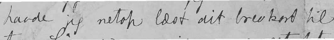havde jeg nætop læst dit brevkort til
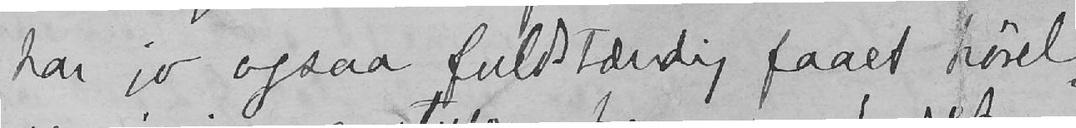har jo ogsaa fuldstændig faaet hørel-
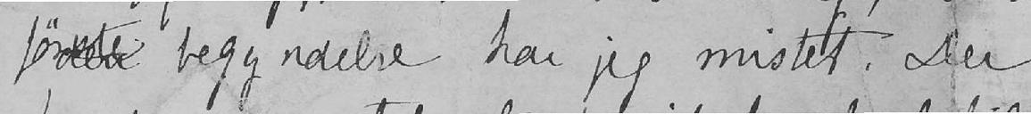første begyndelse har jeg mistet. Der
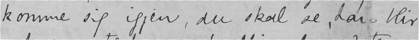komme sig igjen, du skal se, han blir
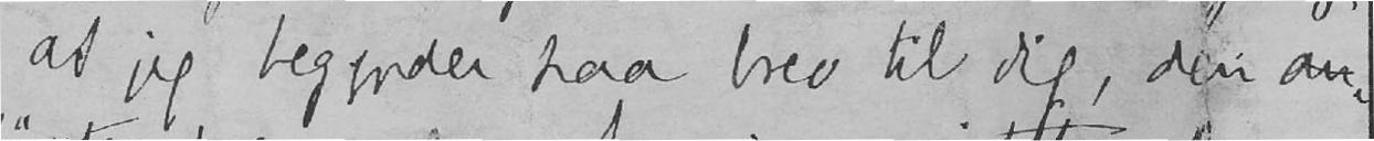at jeg begynder paa brev til dig, den an-
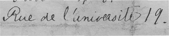Rue de l'université 19.
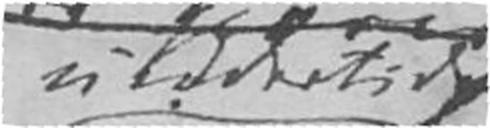undertiden
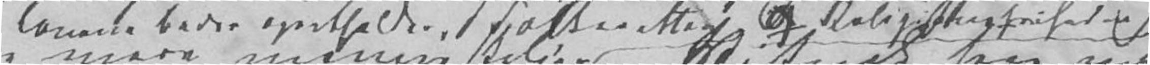Lovene bedre opretholdes, Folkeretten og Religionsfriheden
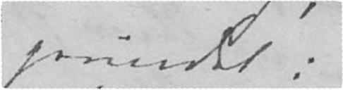grundet i
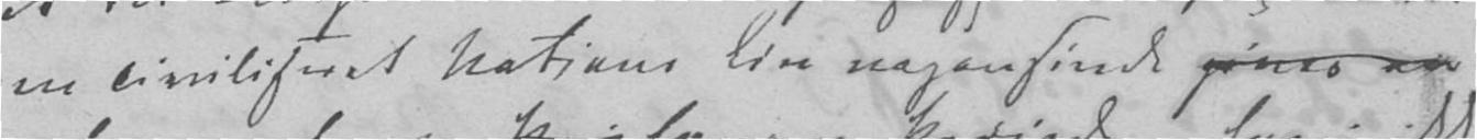en civiliseret Nations Liv nogensinde
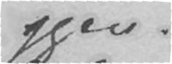gjen-
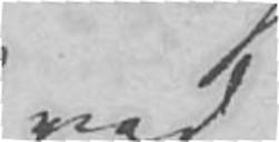ved
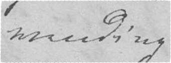vending
